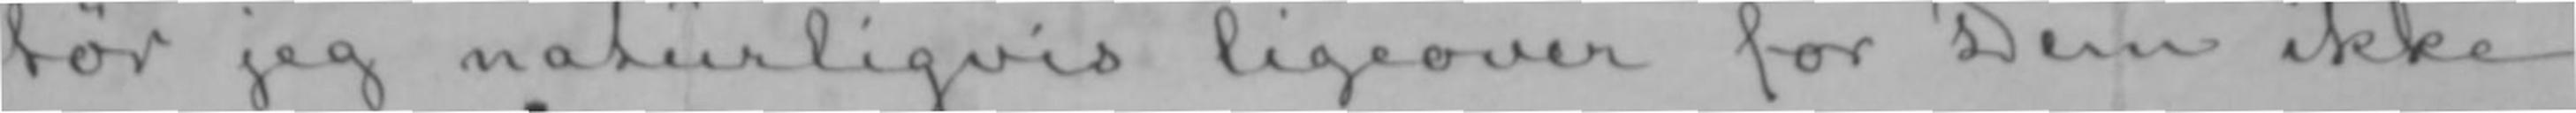tør jeg naturligvis ligeover for Dem ikke
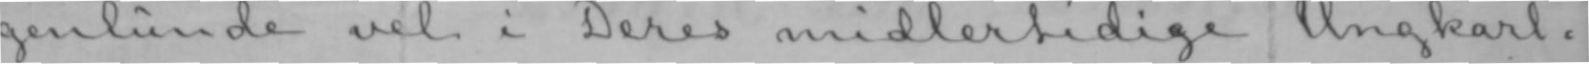genlunde vel i Deres midlertidige Ungkarl-
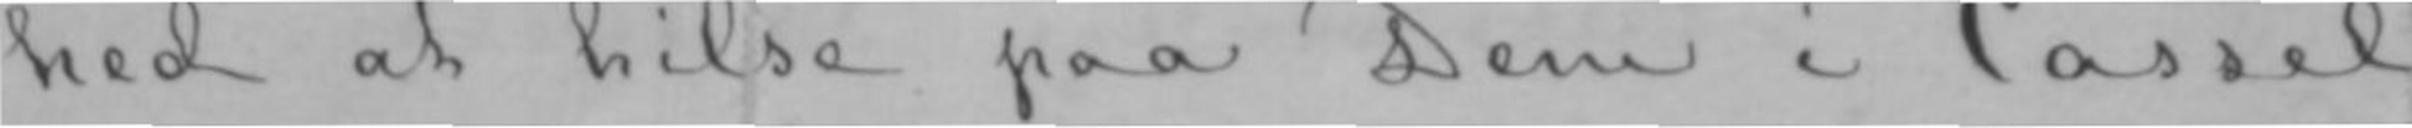hed at hilse paa Dem i Cassel.
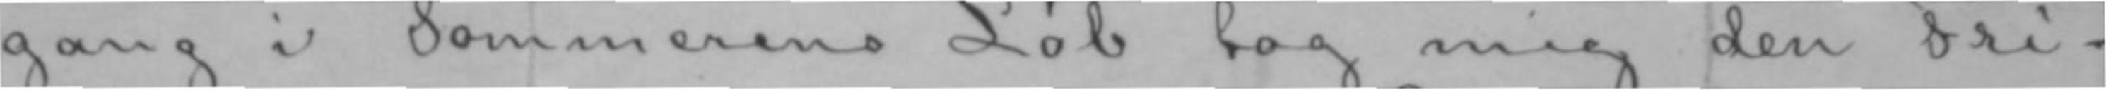gang i Sommerens Løb tog mig den Fri-
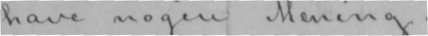have nogen Mening.
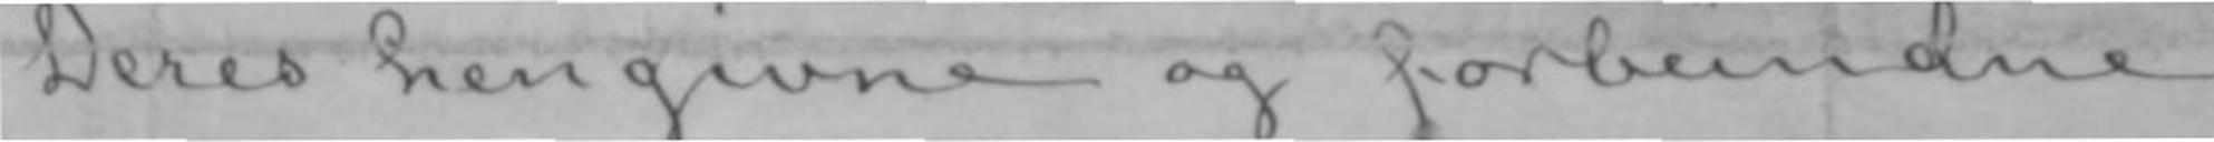Deres hengivne og forbundne
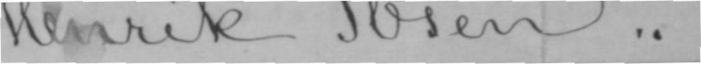Henrik Ibsen..
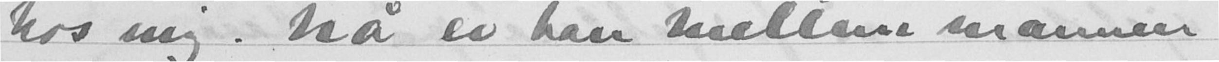hos mig. Nå er han mellom mannen
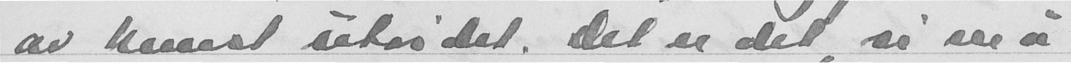av kunst utvidet. Det er det, vi må
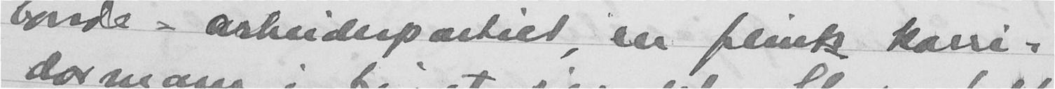bonde-arbeiderpartiet, en flink korri-
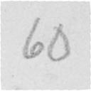60
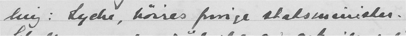ling: Lyche, høires forrige statsminister.
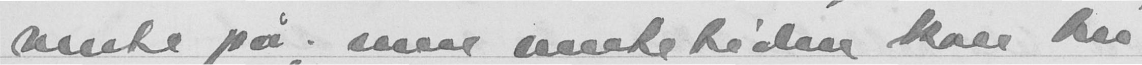vente på, men ventetiden kan bli
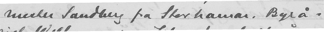mester Sandberg fra Storhamar, Byrå-
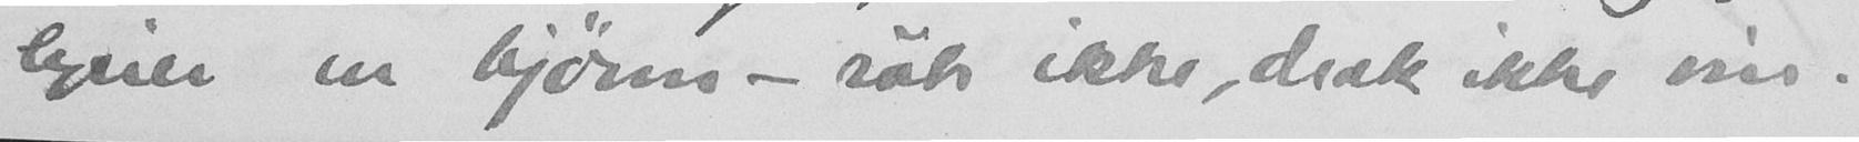ligner en bjørn - røk ikke, drak ikke vin.
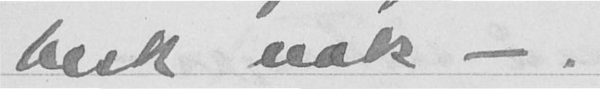besk nok - .
